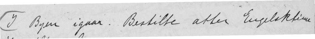I Byen igaar. Bestilte atter Engelsktime
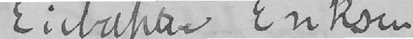Eiebakke, Eriksen,
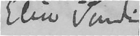Elise Sinding
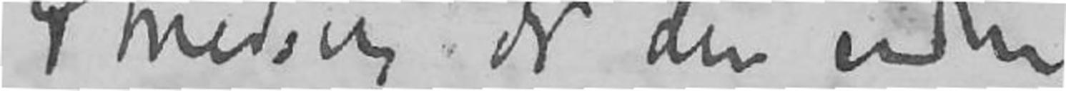Skredsvig er der endnu
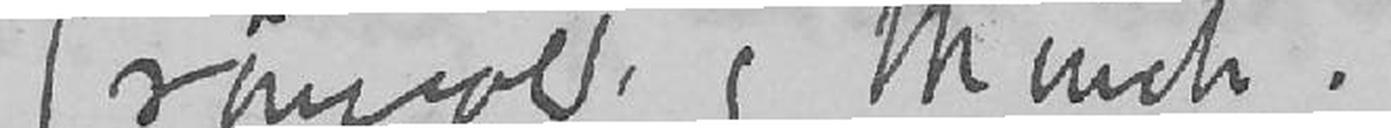Grønvold og Munch.
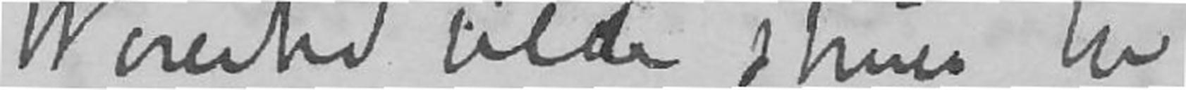Werenskiold vilde skrive til
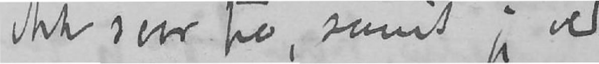ikke svar fra, samt jeg ved
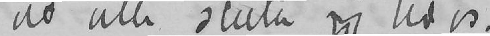at ville slutte sig til os.
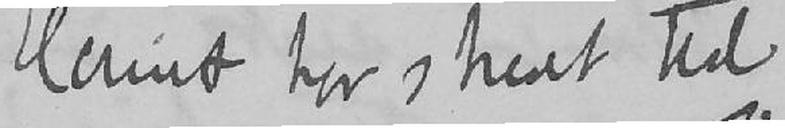Harriet har skrevet til
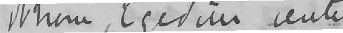Thorne, Egedius ventes
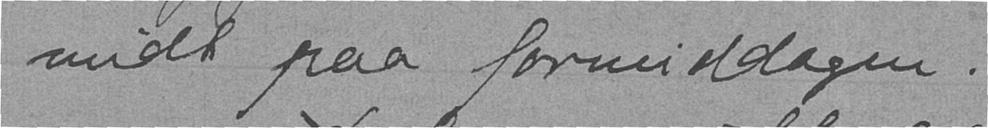midt paa formiddagen.
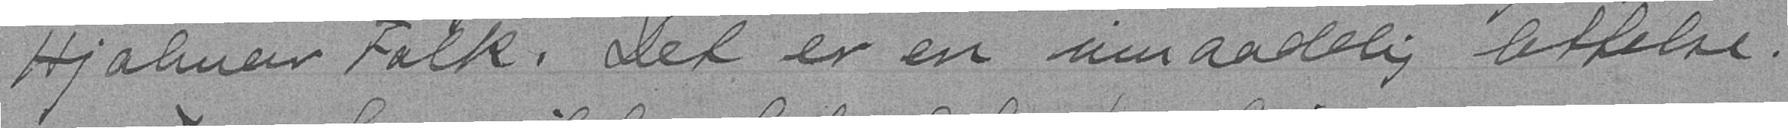Hjalmar Falk. Det er en umaadelig lettelse.
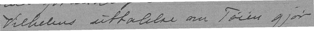Vilhelms uttalelse om Tøien gjør
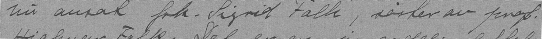nu ansat frk. Sigrid Falk, søster av prof.
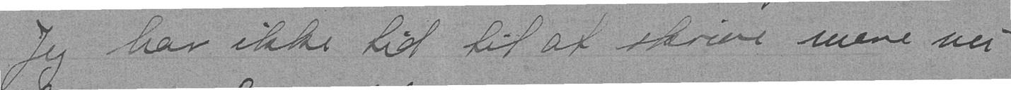Jeg har ikke tid til at skrive mere nu
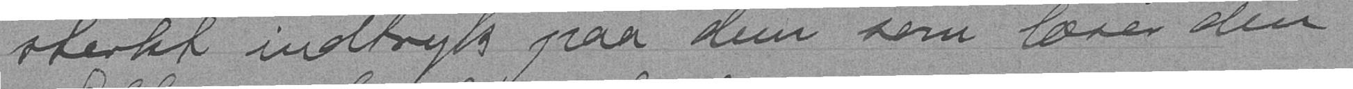sterkt indtryk paa dem som læser den.
